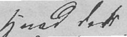Hvad det
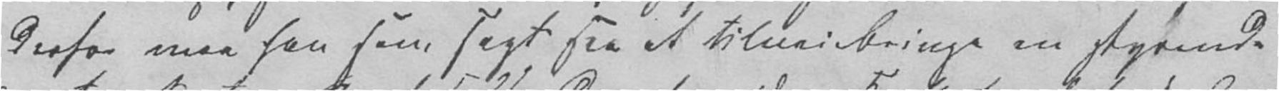Derfor maa han som sagt see at tilveiebringe en styrende
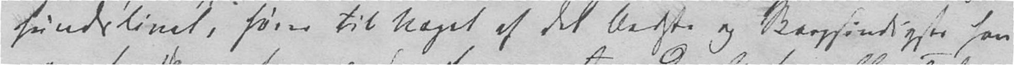fundslivet, hører til Noget af det bedste og Skarpsindigste han
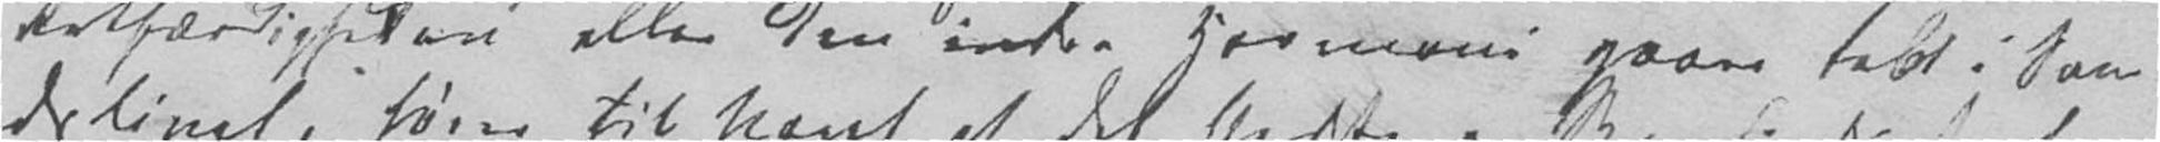Retfærdighedens eller den indre Harmoni gaaer tabt i Sam-
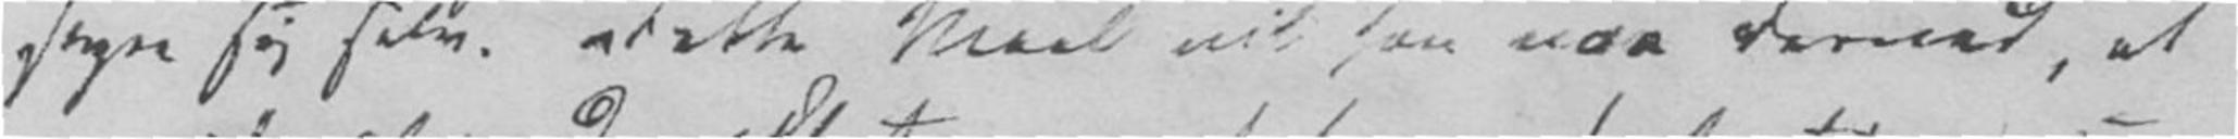styre sig selv. Dette Maal vil han naa derved, at
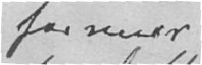formenes
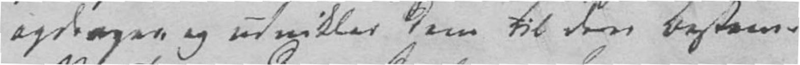opdrager og udvikler dem til deres Bestem-
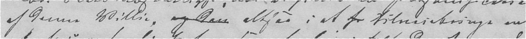af denne Villie, og den altsaa i at fr tilveiebringe en
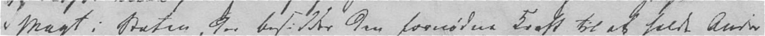Magt i Staten, der besidder den fornødne Kraft til at holde Andre
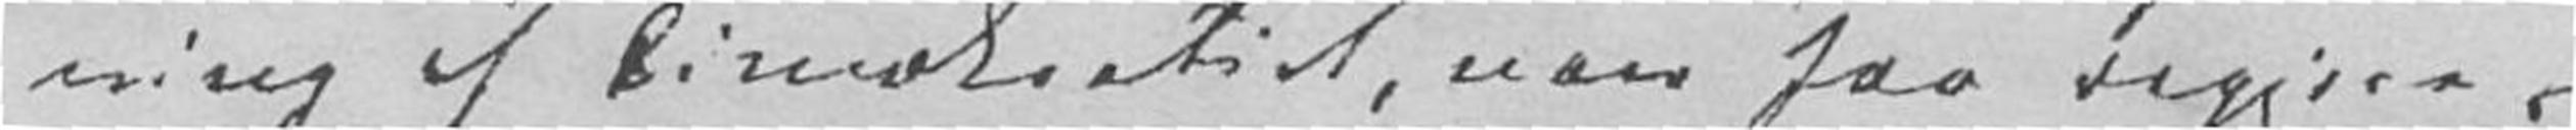ning af Timokratiet, naar faa regjere,
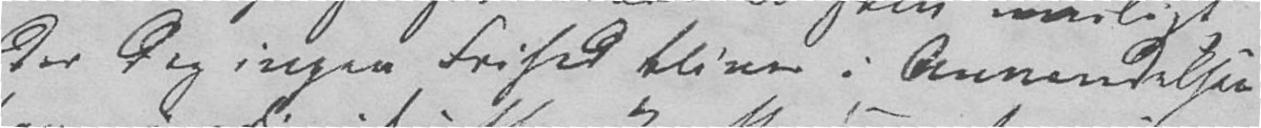der dog ingen Frihed bliver i Anvendelsen
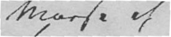Masse af
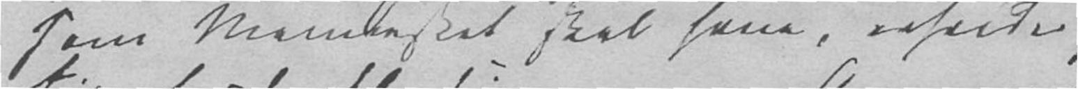som Mennesket skal have, erholder
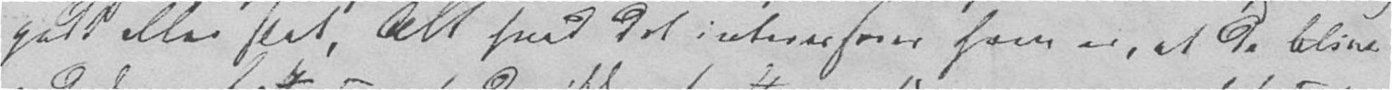godt eller slet, Alt hvad det interesserer ham er, at de blive
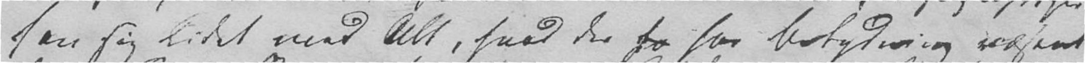han sig lidet med Alt, hvad der fo har Betydning væsent-
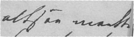altsaa maatte
